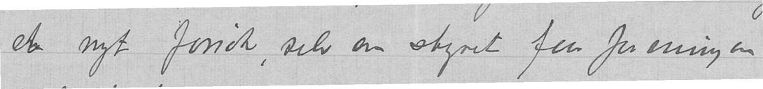et nyt forsøk, selv om styret faar foreningen
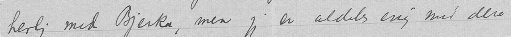herlig med Bjerke, men jeg er aldeles enig med dere
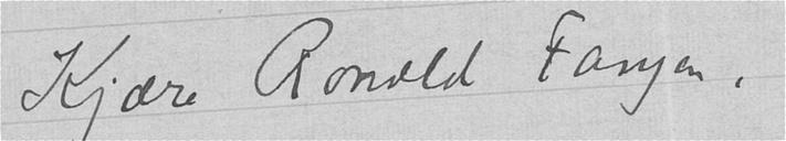Kjære Ronald Fangen,
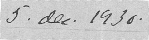5. dec. 1930.
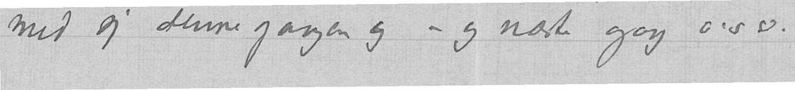med sig denne gangen og – og næste gang o.s v.
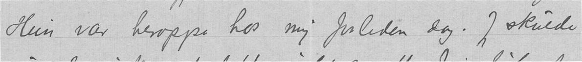Hun var heroppe hos mig forleden dag. Jeg skulde
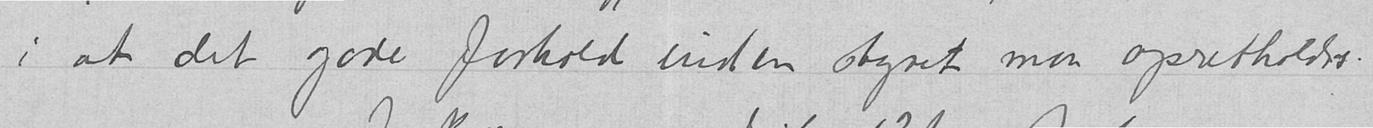i at det gode forhold inden styret maa opretholdes.
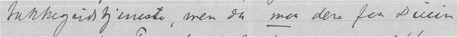takkegudstjeneste, men da maa dere faa Duun
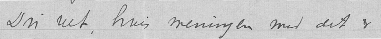Du vet, hvis meningen med det er
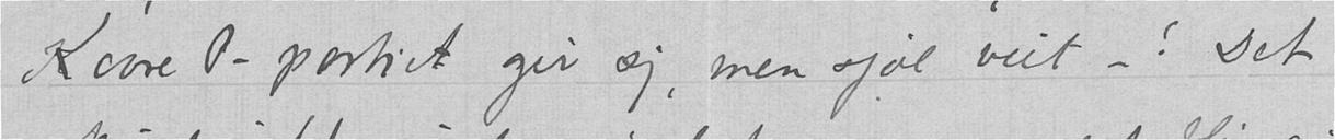Kaare P-partiet gir sig, men sjøl veit – ! Det
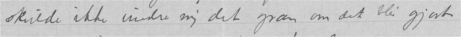skulde ikke undre mig det gran om det blir gjort
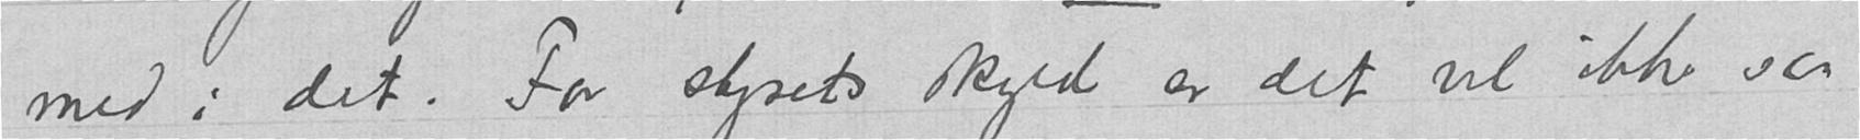med i det. For styrets skyld er det vel ikke saa
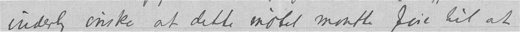inderlig ønske at dette møtet maatte føre til at
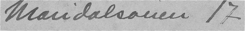Maridalsveien 17
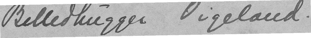Billedhugger Vigeland.
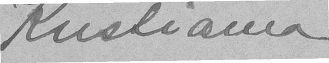Kristiania
